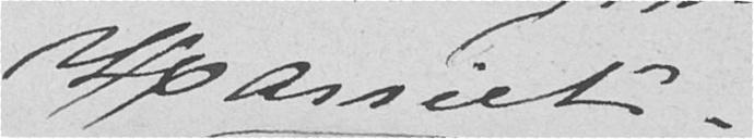Harriet.
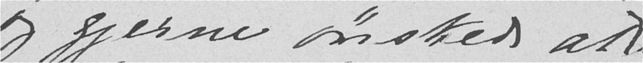og gjerne ønsked at
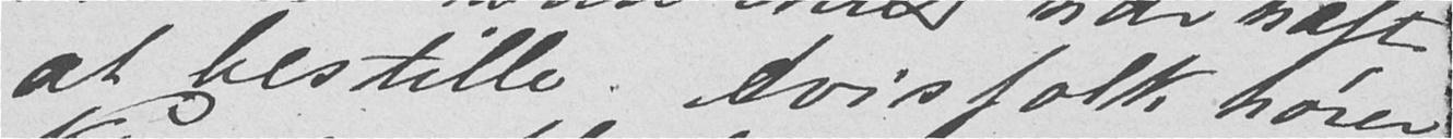at bestille. Avisfolk hører
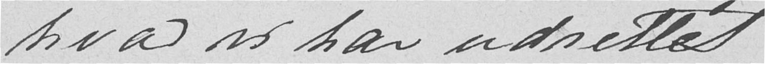hvad vi har udrettet og
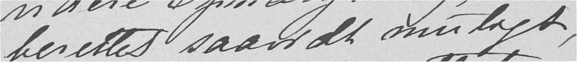berettet saa vidt muligt,
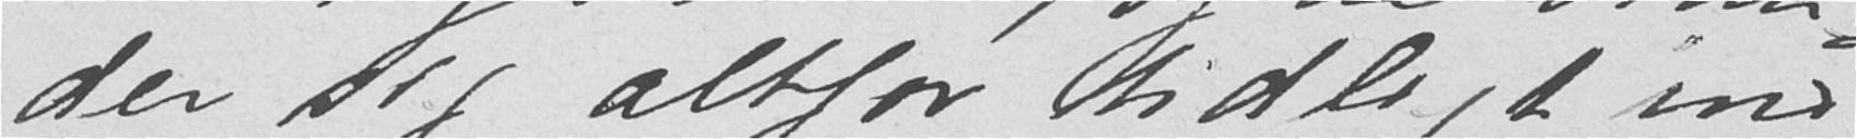der sig altfor tidligt ind
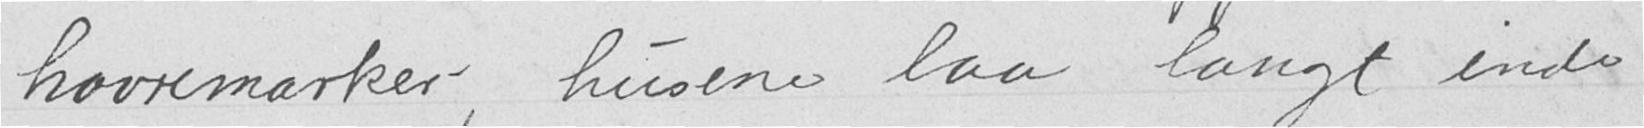havremarker, husene laa langt inde
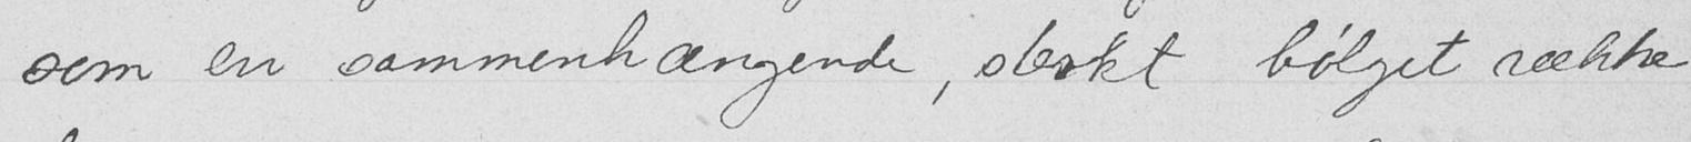som en sammenhængende, sterkt bølget række
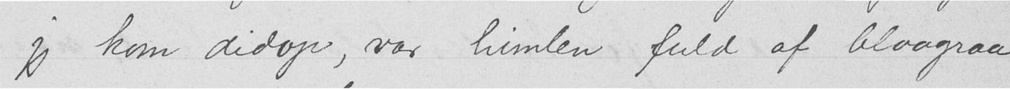jeg kom didop, var himlen fuld af blaagraa
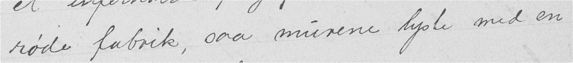røde fabrik, saa murene lyste med en
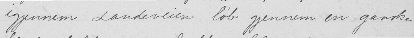igjennem. Landeveien løb gjennem en ganske
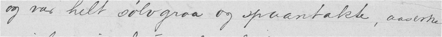og var helt sølvgraa og spaantækte, aaserne
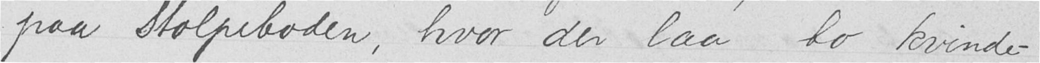paa stolpeboden, hvor der laa to kvinde-
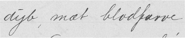dyb, mæt blodfarve — —.
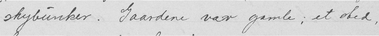skybunker. Gaardene var gamle; et sted,
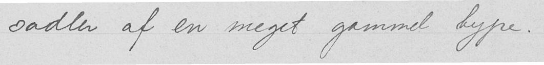sadler af en meget gammel type.
